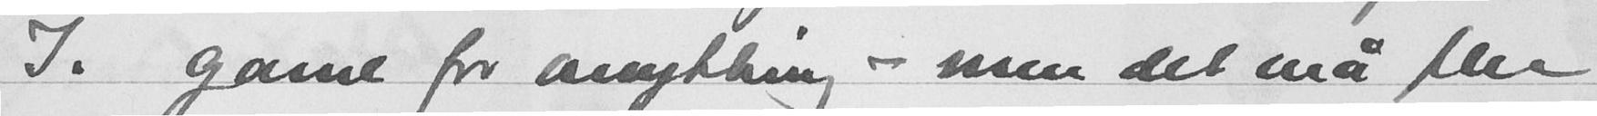J. game for anrykking - men det må fler
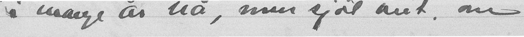i mange år nå, men sjål krit, om
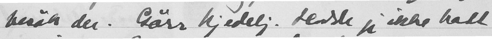besøk der. Gørr kjedelig. Hadde jeg ikke hatt
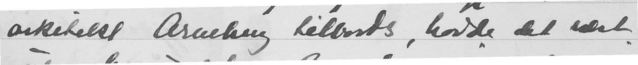arkitekt Arneberg tilbords, hadde det vært
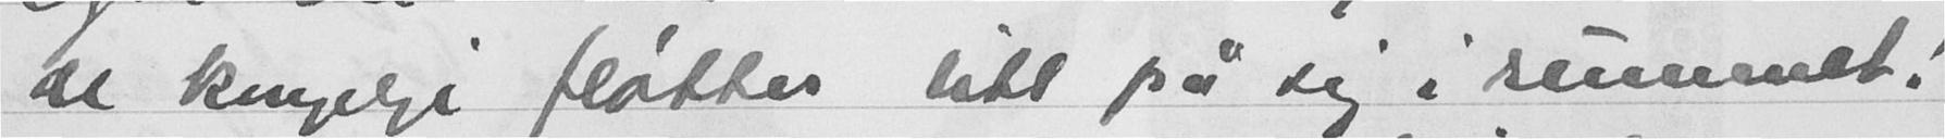de kongelige flåtter litt på sig i rummet!
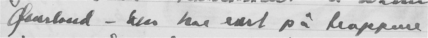Øverland - han har vært pa trappene
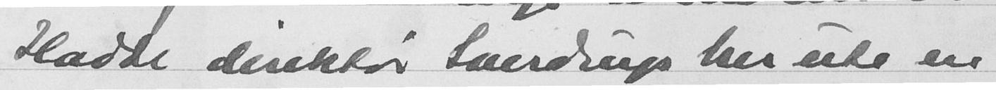Hadde direktør Sverdrups her ute en
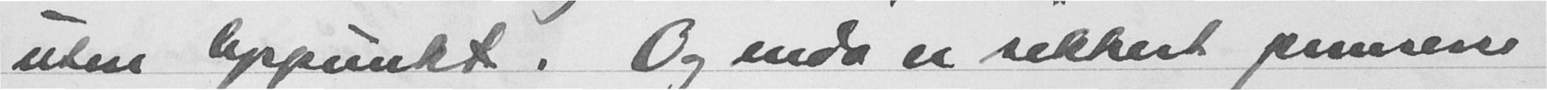uten lyspunkt. Og enda er sikkert prinsens
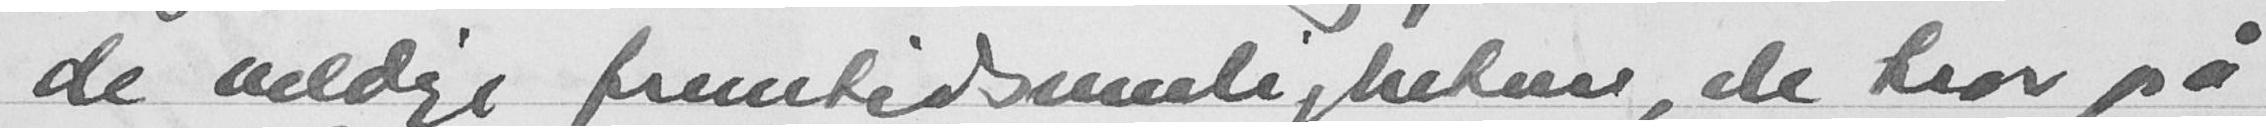de veldige fremtidsmulighetene, de tror på
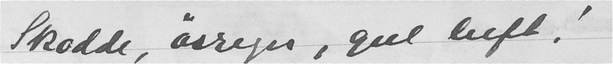Skodde, øsregn, gul luft!
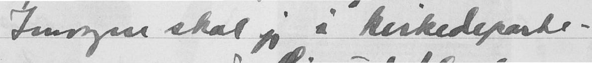Imorgen skal jeg i kirkedeparte-
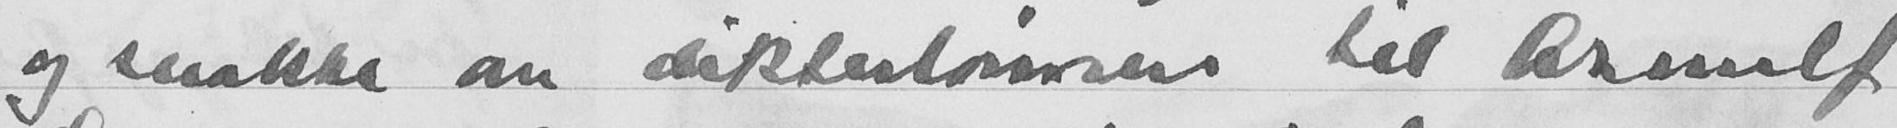og snakke om dikterlønnen til Arnulf
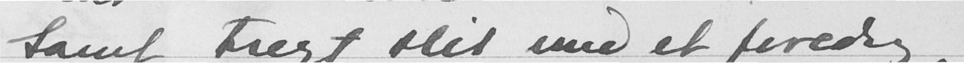Samt tregt slit med et foredrag,
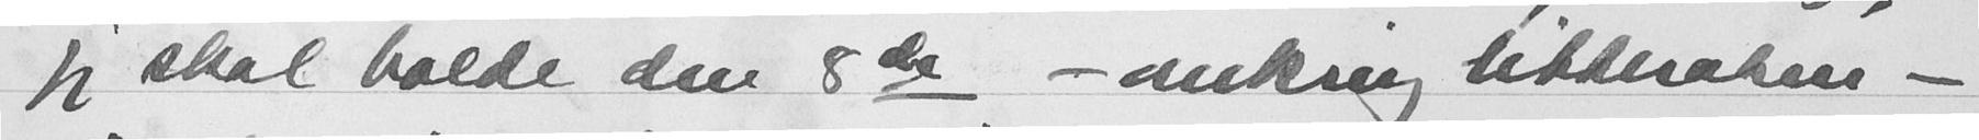jeg skal holde den 8de, - omkring litteraturen -
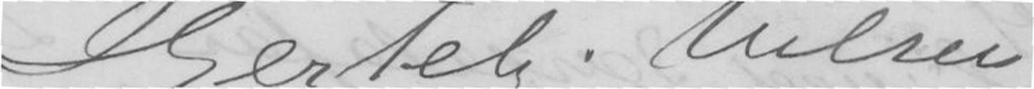Hjertelig hilsen
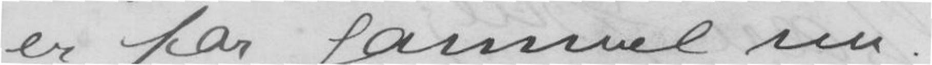er for gammel nu.
